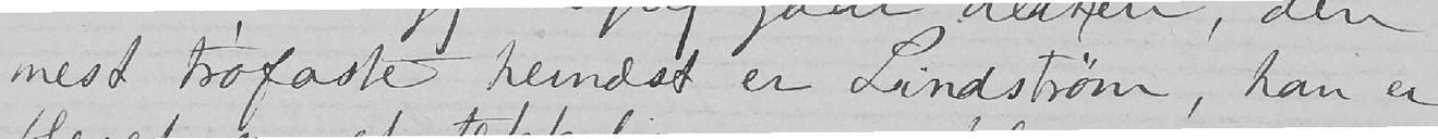mest trofaste hernæst er Lindstrøm, han er
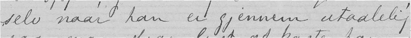selv naar han er gjennem utaalelig,
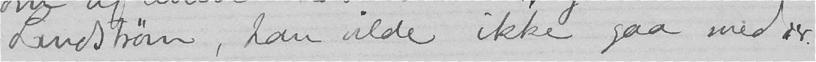Lindstrøm, han vilde ikke gaa med der.
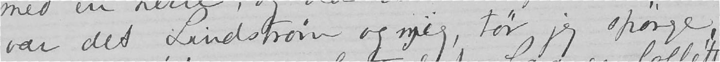var det Lindstrøm og mig, tør jeg spørge,
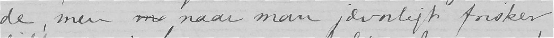de, men m naar man jævnligt frisker
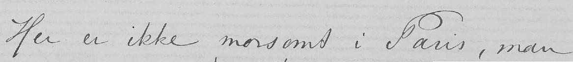Her er ikke morsomt i Paris, man
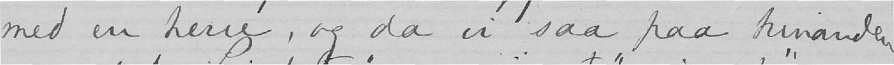med en herre, og da vi saa paa hinanden
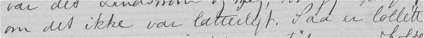om det ikke var latterligt. Saa er Collett
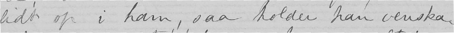lidt op i ham, saa holder han venska-
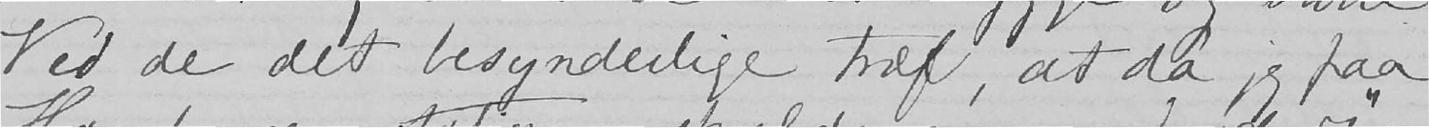Ved de det besyndelige træf, at da jeg paa
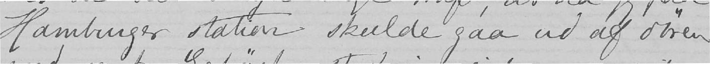Hamburger station skulde gaa ud af døren
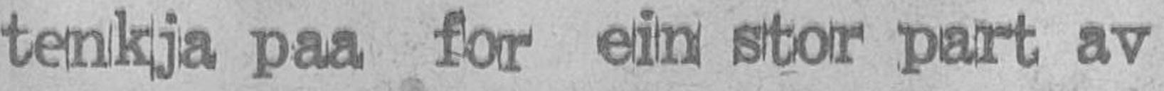tenkja paa for ein stor part av
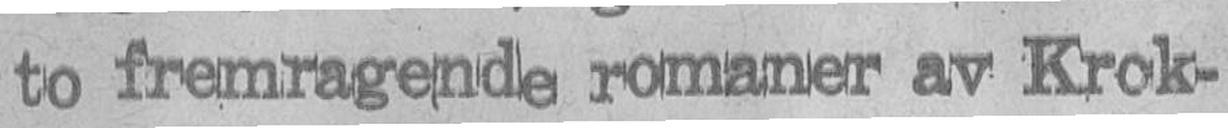to fremragende romaner av Krok-
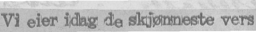Vi eier idag de skjønneste vers
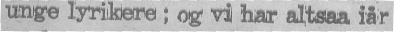unge lyrikere; og vi har altsaa iår
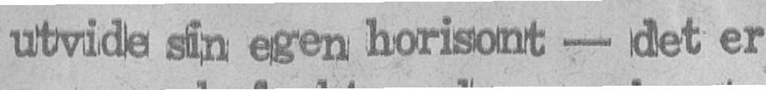utvide sin egen horisont — det er
